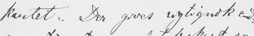kartet. Der gives rigtignok end-
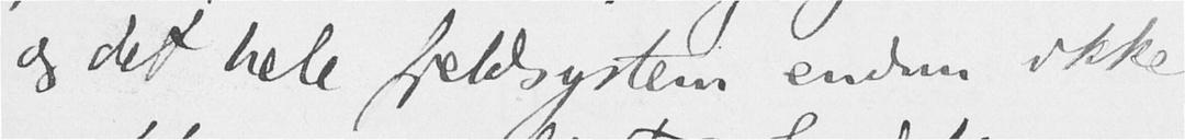og det hele fjeldsystem endnu ikke
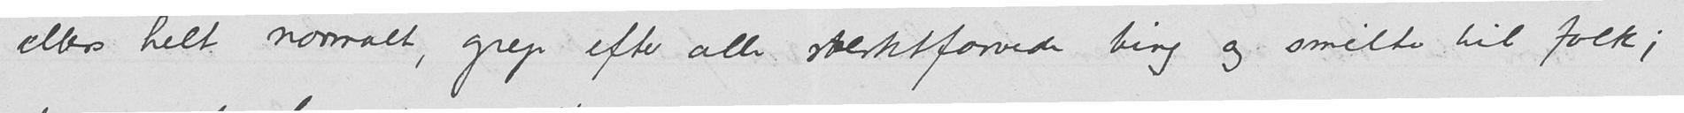ellers helt normalt, grep efter alle sterktfarvede ting og smilte til folk;
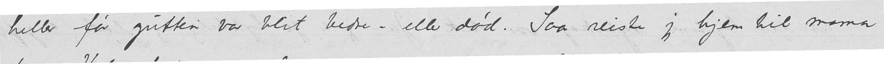heller før gutten var blit bedre - eller død. Saa reiste jeg hjem til mama
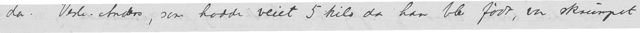da. Vesle-Anders, som hadde veiet 5 kilo da han ble født, var skrumpet
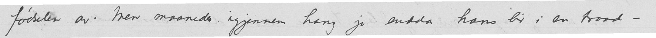fødselen av. Men maaneder igjennem hang jo endda hans liv i en traad -
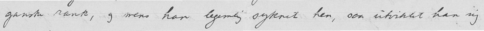ganske rank, og mens han legemlig syknet hen, saa utviklet han sig
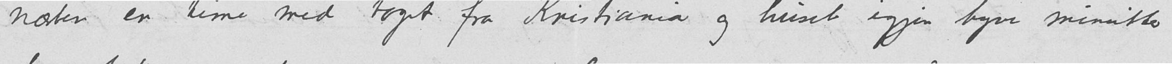næsten en time med toget fra Kristiania og huset igjen tyve minutter
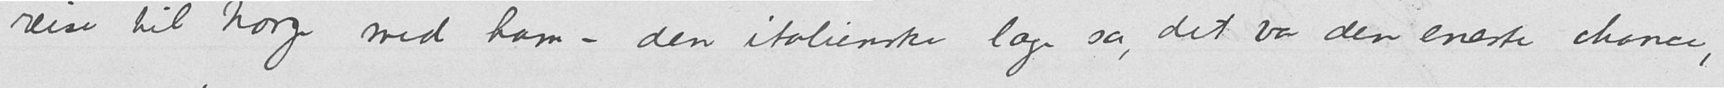reise til Norge med ham - den italienske lege sa, det var den eneste chance,
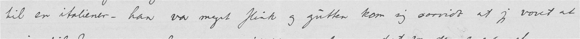til en italiener - han var meget flink og gutten kom sig saavidt at jeg vovet at
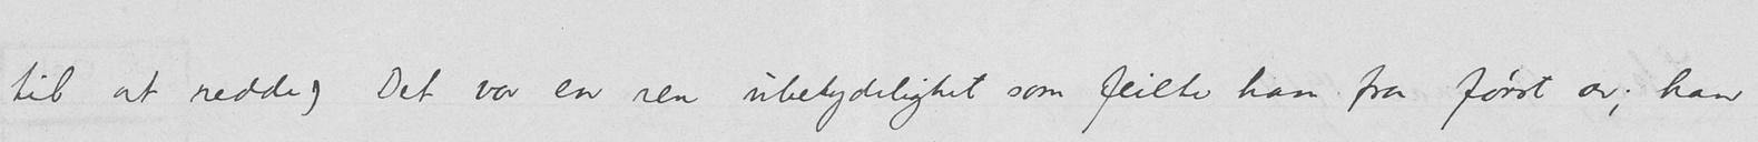til at redde) Det var en ren ubetydelighet som feilte han fra først av; han
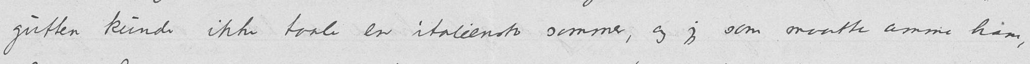gutten kunde ikke taale en italiensk sommer, og jeg som maatte amme han,
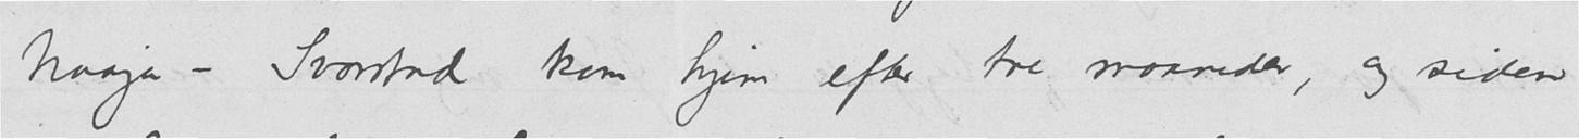Naaja - Svarstad kom hjem efter tre maaneder, og siden
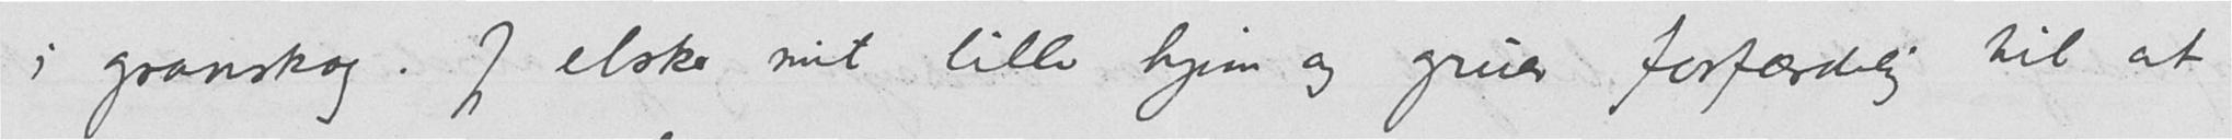i granskog. Jeg elsker mit lille hjem og gruer forfærdelig til at
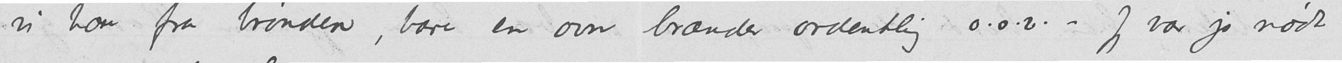vi bære fra brønden, bare en ovn brænder ordentlig o.s.v. Jeg var jo nødt
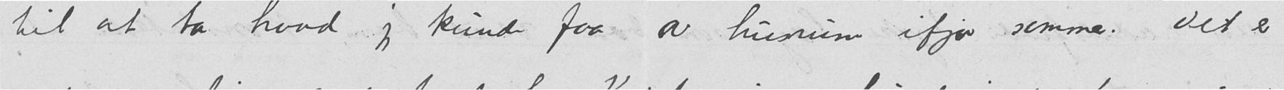til at ta hvad jeg kunde faa av husrum ifjor sommer. Det er
